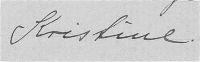Kristine.
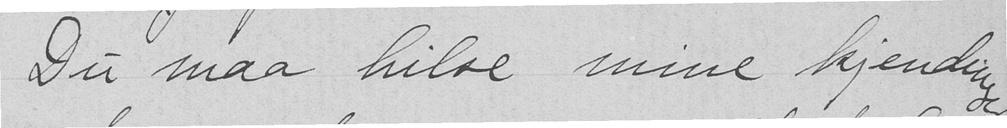Du maa hilse mine kjendinger
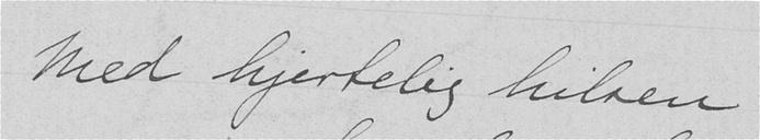Med hjertelig hilsen
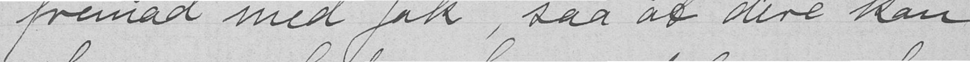fremad med Jak, saa at dere kan
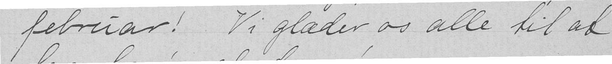februar! Vi glæder os alle til at
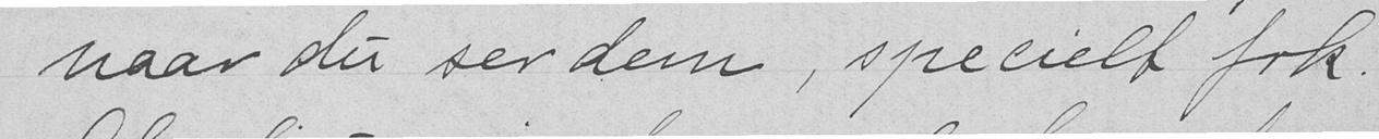naar du ser dem, specielt frk.
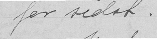for sidst.
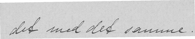det med det samme.
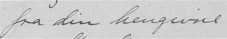fra den hengivne
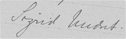Sigrid Undset.
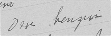Deres hengivne
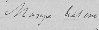Mange hilsener
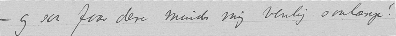- og saa faar dere mindes mig venlig saalænge!
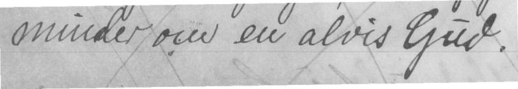minder om en alvis Gud.
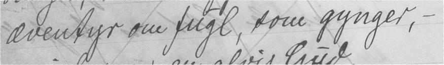æventyr om fugl, som gynger, -
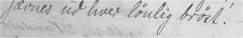jævnes ud hver lønlig brøst. -
